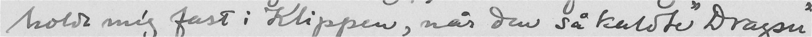holde mig fast i Klippen, når den såkaldte "Dragsu"
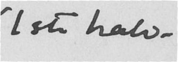1ste halv-
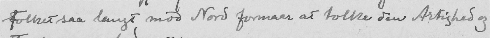Folket saa langt mod Nord formaar at tolke den Artighed og
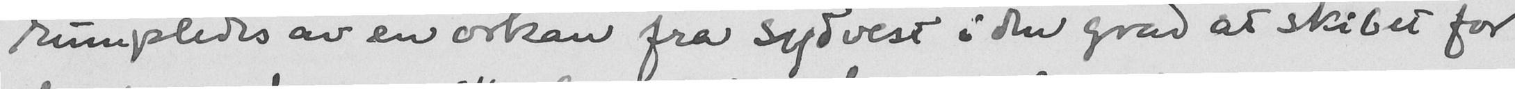rumpledes av en orkan fra sydvest i den grad at skibet for
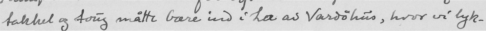takkel og toug måtte bære ind i læ av Vardøhus, hvor vi lyk-
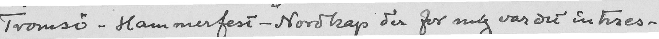Tromsø - Hammerfest - "Nordkap der for mig var det interes-
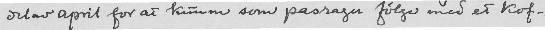del av april for at kunne som passager følge med et kof-
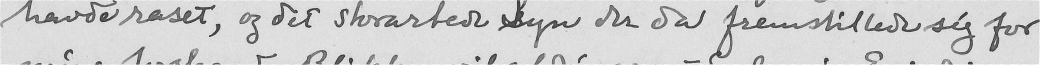havde raset, og det storartede syn der da fremstillede sig for
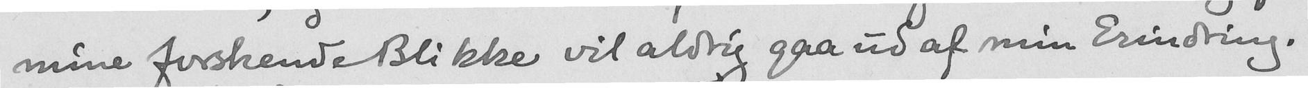mine forskende Blikke vil aldrig gaa ud af min Erindring.
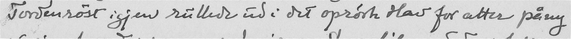Tordenrøst igjen rullede ud i det oprørte Hav for atter påny
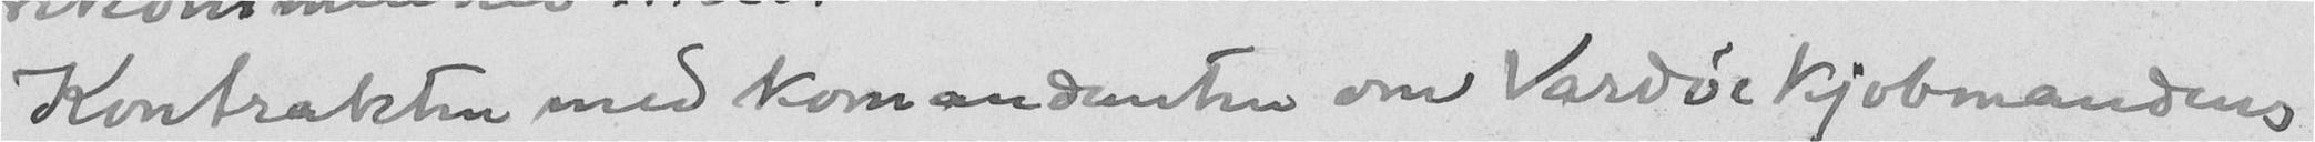Kontrakten med komandanten om Vardøekjøbmandens
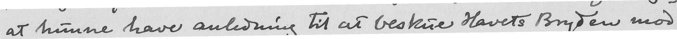at kunne have anledning til at beskue Havets Bryden mod
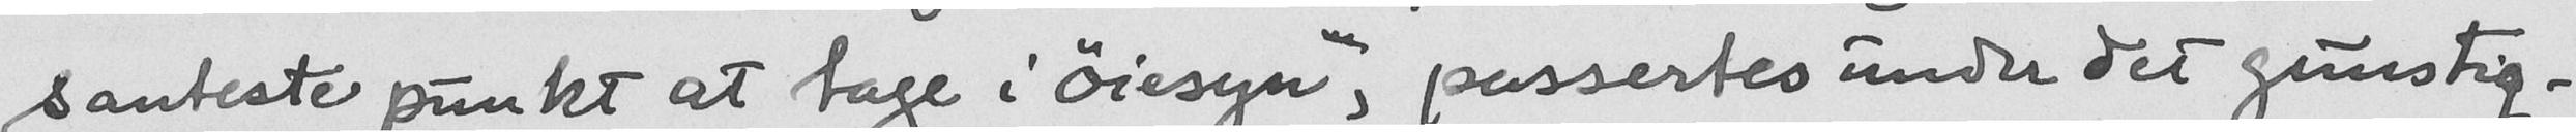santeste punkt at tage i øiesyn", passertes under det gunstig-
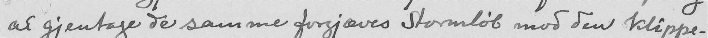at gjentage de samme forgjæves Stormløb mod den klippe-
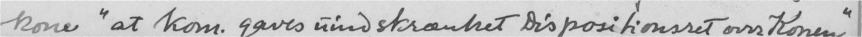kone "at kom. gaves uindskrænket Dispositionsret over Konen."
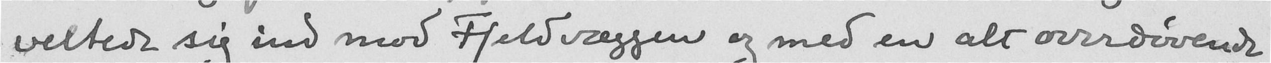veltede sig ind mod Fjeldvæggen og med en alt overdøvende
